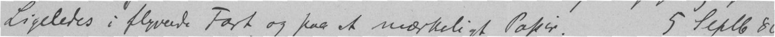Ligeledes i flyvende Fart og paa et mærkeligt Papir. 5 Septb. 86
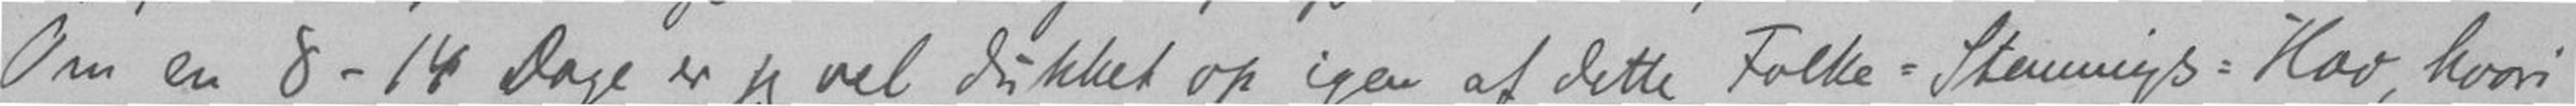Om en 8-14 Dage er jeg vel dukket op igen af dette Folke-Stemnings-Hav, hvori
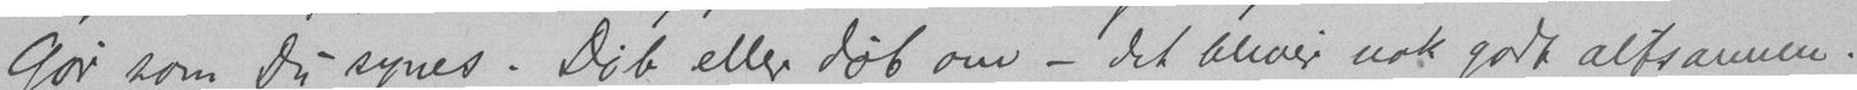Gør som Du synes. Døb eller Døb om - det bliver nok godt altsammen.
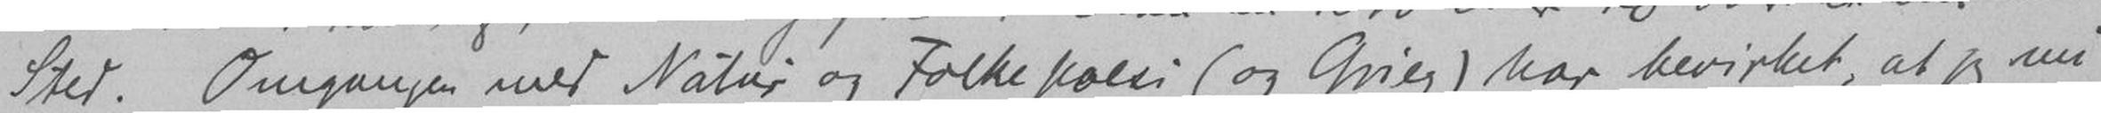Sted. Omgangen med Natur og Folkepoesi (og Grieg) har bevirket, at jeg nu
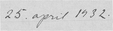25. april 1932.
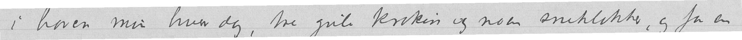i haven min hver dag, tre gule krokus og noen sneklokker, og for en
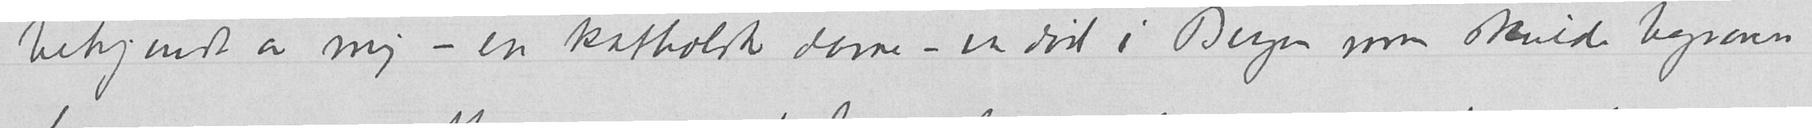bekjendt av mig - en katholsk dame - var død i Bergen som skulde begraves
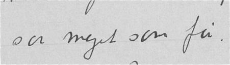saa meget som før.
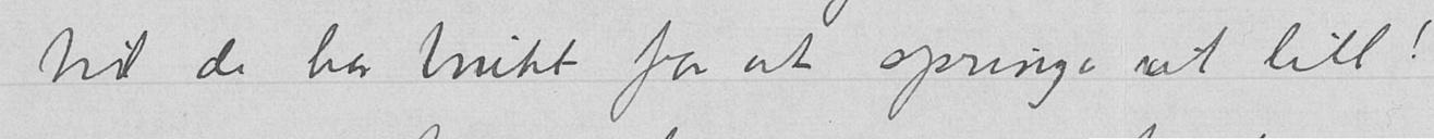tid de har brukt for at springe ut litt!
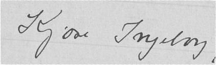Kjære Ingeborg,
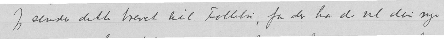Jeg sender dette brevet til Follebu, for der har de vel din nye
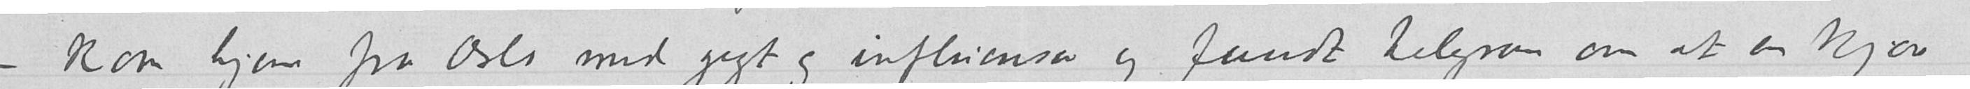- kom hjem fra Oslo med gigt og influensa og fandt telegram om at en kjær
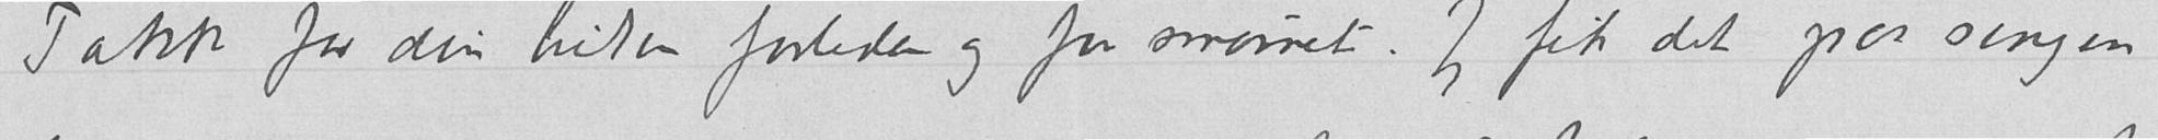Takk for din hilsen forleden og for smørret. Jeg fik det paa sengen
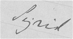Sigrid
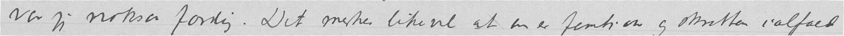var jeg noksaa færdig. Det merkes likevel at en er femti aar og skrotten ialfald
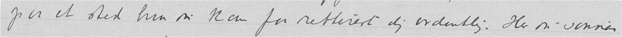paa et sted hvor du kan faa rettirert dig ordentlig. Har du sønnen
